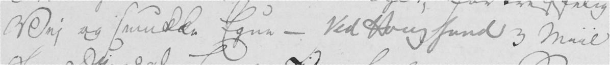Vej og smukke Egne - Ved Hougsund 3 Miil
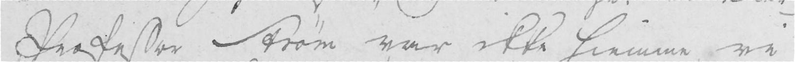Professor Strøm var ikke hiemme, vi
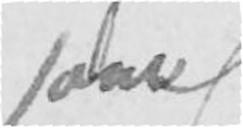som
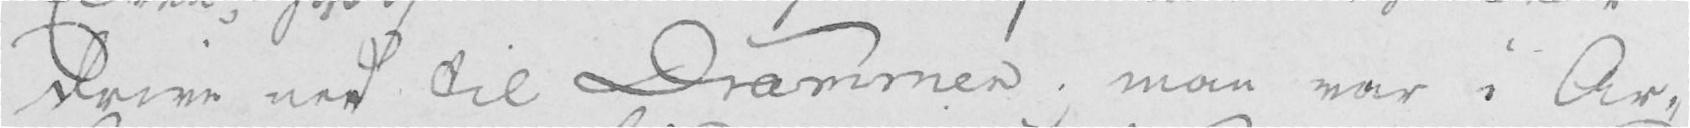drive ned til Drammen; man var i Ar-
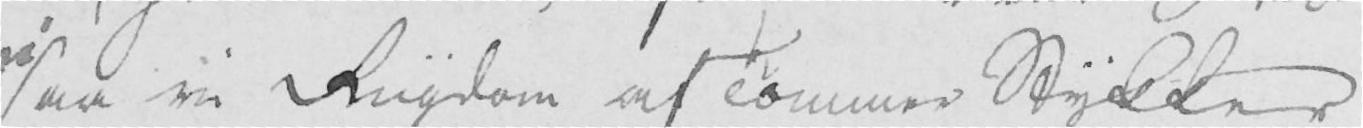saa vi Riigdom af Tømmer Stykker
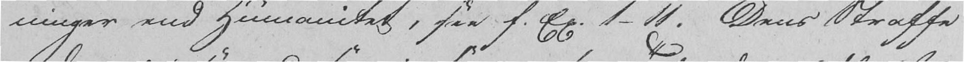ninger end Humanitet, see f. Ex. 1-11. Dens Straffe
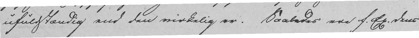ufuldstændig end den virkelig er. Saaledes ere f. Ex. dens
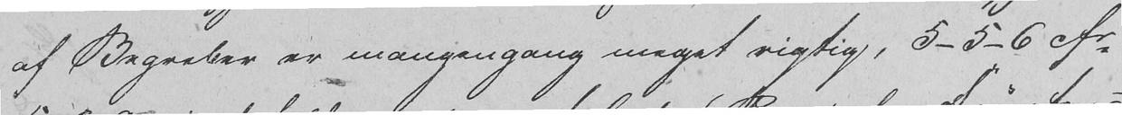af Begreber er mangengang meget rigtig, 5-5-6 cfr
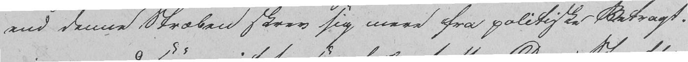end denne Stræben skrev sig mere fra politiske Betragt-
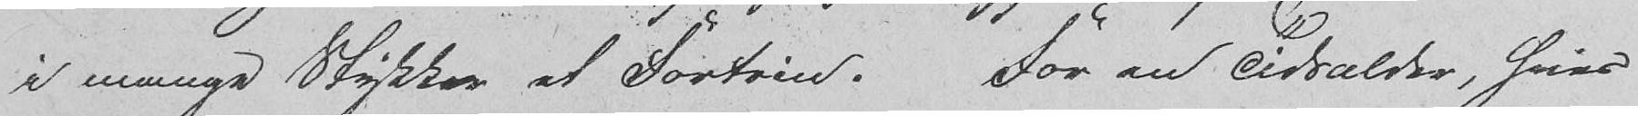i mange Stykker et Fortrin. For en Tidsalder, hvis
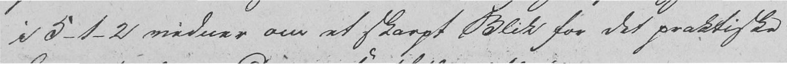i 5-1-2 vidner om et skarpt Blik for det praktiske
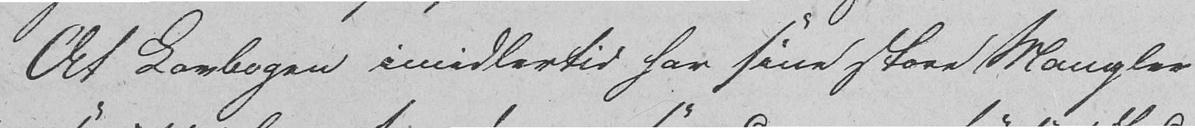At Lovbogen imidlertid har sine store Mangler
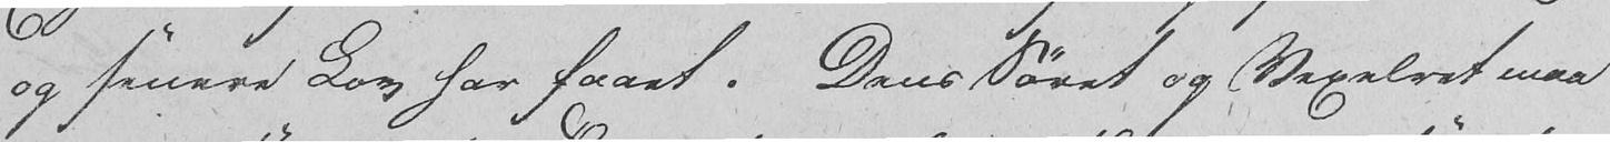og senere Lov har faaet. Dens Søret og Vexelret maa
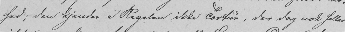hed; den kjender i Regelen ikke Tortur, der dog nok heller
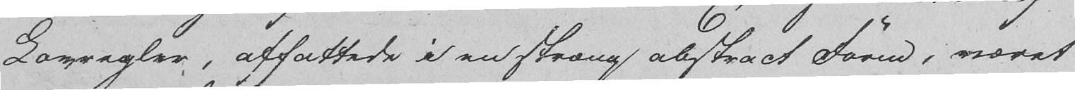Lovregler, affattede i en stræng abstract Form, været
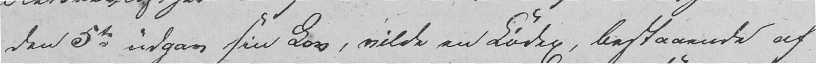den 5te udgav sin Lov, vilde en Kodex, bestaaende af
